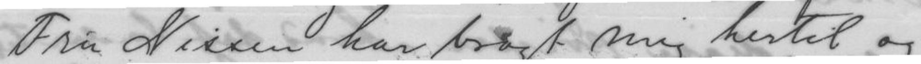Fru Nissen har bragt mig hertil og
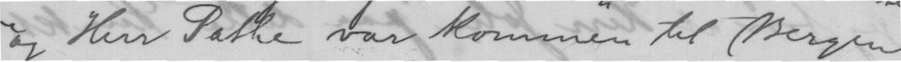og Herr Pathe var kommen til Bergen
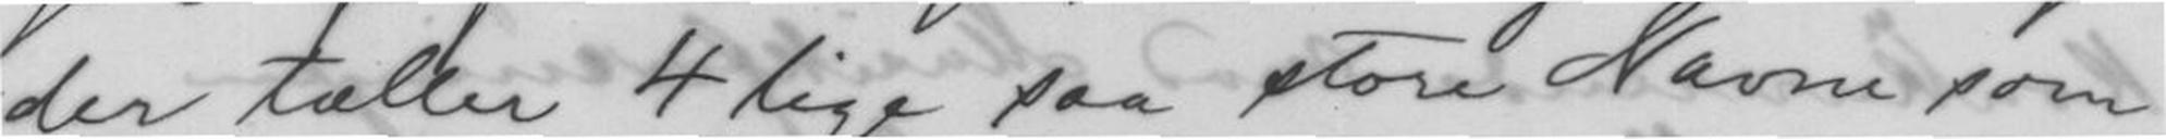der tæller 4 lige saa store Navne som
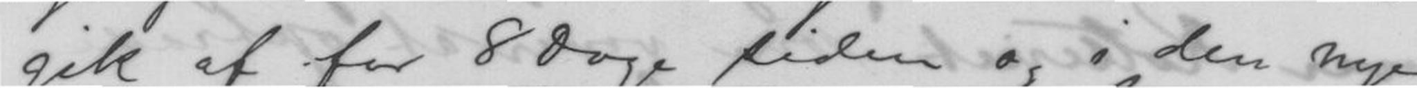gik af for 8 Dage siden og i den nye
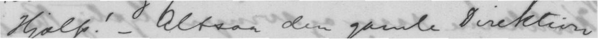Hjælp! - Altsaa den gamle Direktion
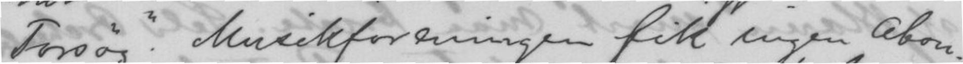Forsøg. Musikforeningen fik ingen Abon
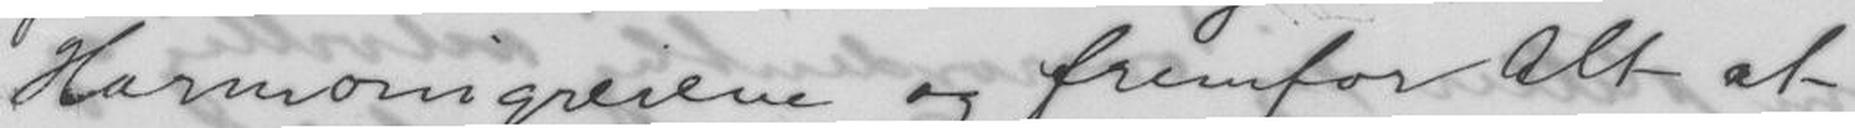Harmonigreiene og fremfor Alt at
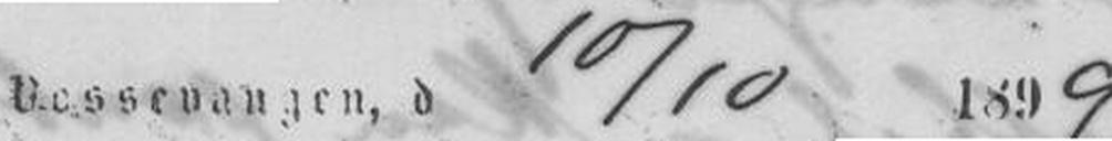Vossevangen, d 10/10 1899
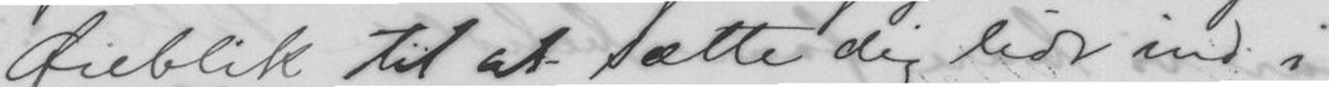Øieblik til at sætte dig lidt ind i
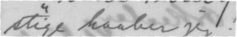stige haaber jeg!
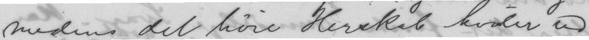medens det høie Herskab hviler ud
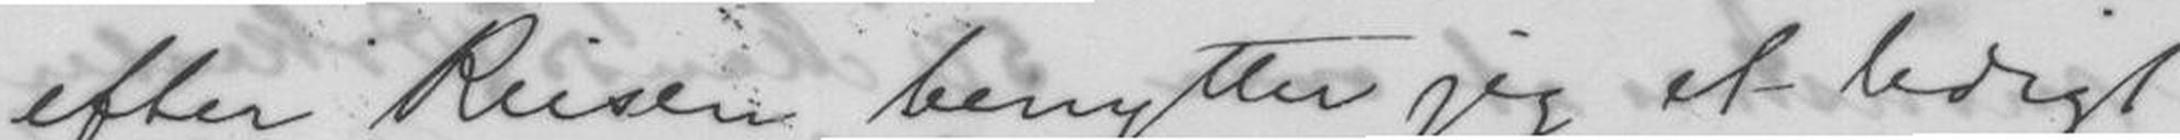efter Reisen benytter jeg et ledigt
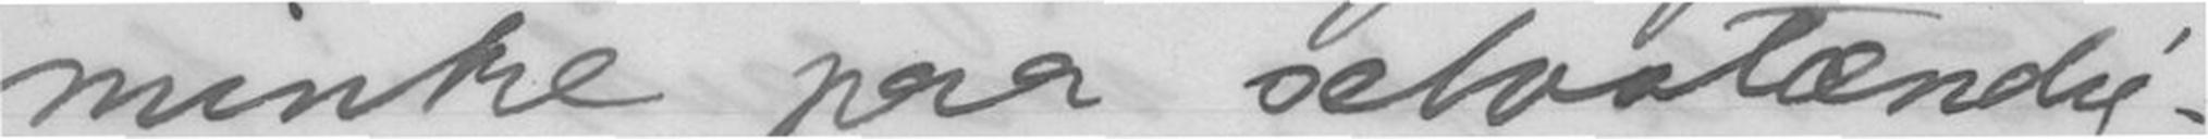minke paa selvslændig-
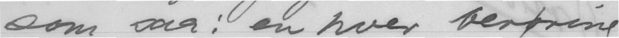som saa: en hver berøring
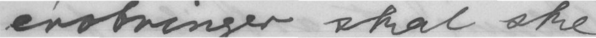erobringer skal ske
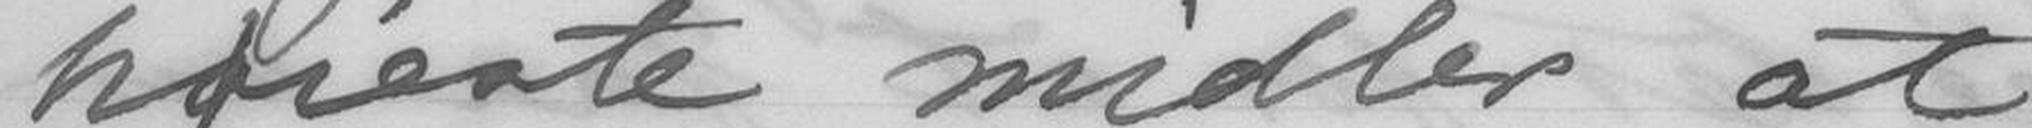høieste midler at
